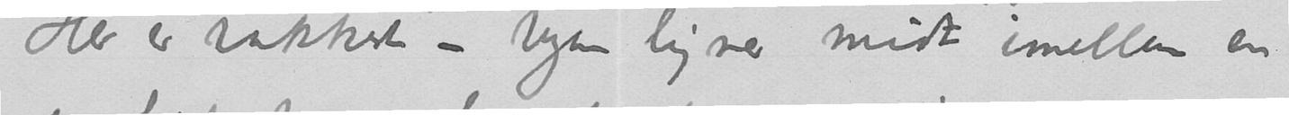Her er vakkert – byen ligner midt imellem en
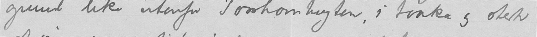grund like utenfor Torshavnbugten, i taake og sterk
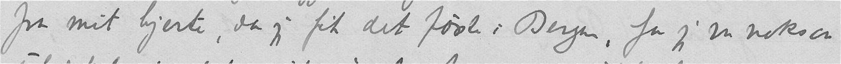fra mit hjerte, da jeg fik det første i Bergen, for jeg var noksaa
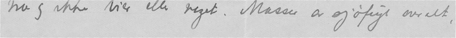træ og ikke vier eller noget. Masser av sjøfugt over alt,
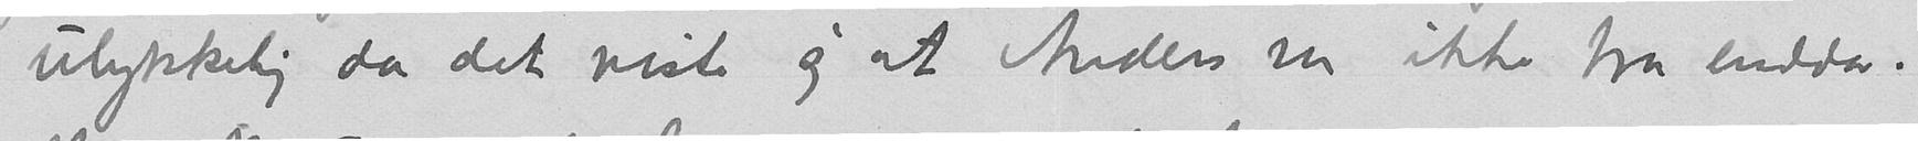ulykkelig da det viste sig at Anders var ikke bra endda.
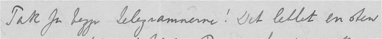Tak for begge telegrammerne! Det lettet en sten
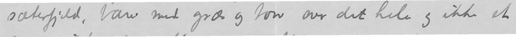sæterfjeld, bare med græs og torv over det hele og ikke et
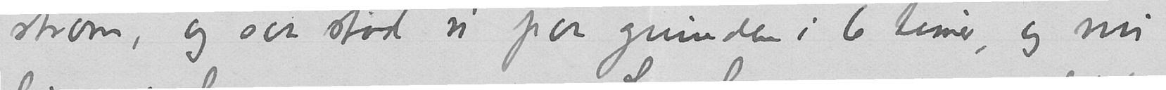strøm, og saa stod vi paa grunden i 6 timer, og nu
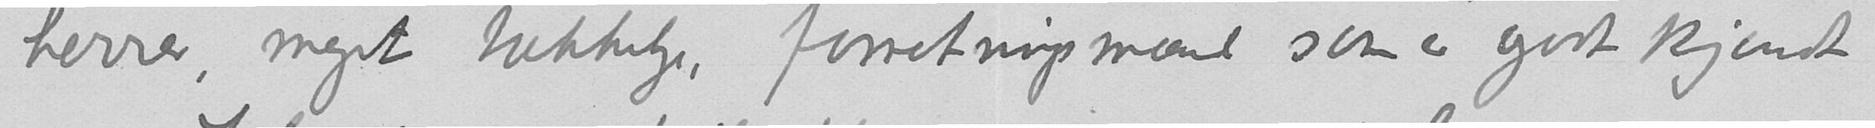herrer, meget tækkelige, forretningsmænd som er godt kjendt
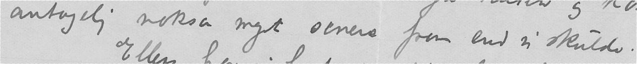antagelig noksaa meget senere frem end vi skulde.
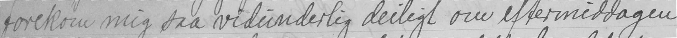forekom mig saa vidunderlig deiligt om eftermiddagen
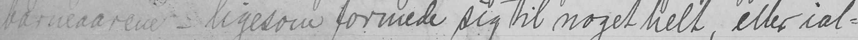barneaarene - ligesom formede sig til noget helt, eller ial-
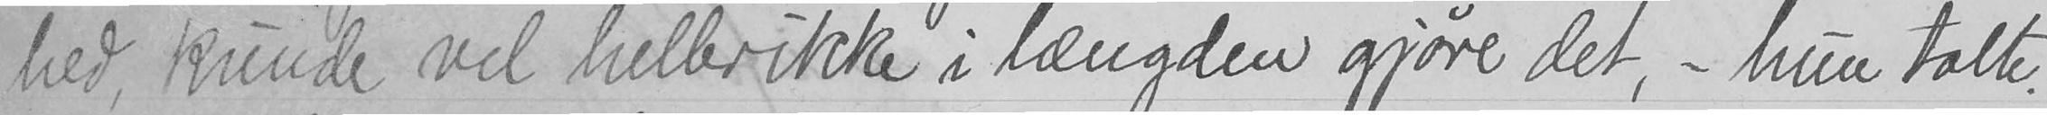hed, kunde vel hellerikke i længden gjøre det, - hun talte
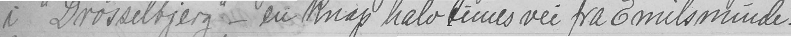i "Drøsselbjerg" - en knap halv times vei fra Emilsminde.
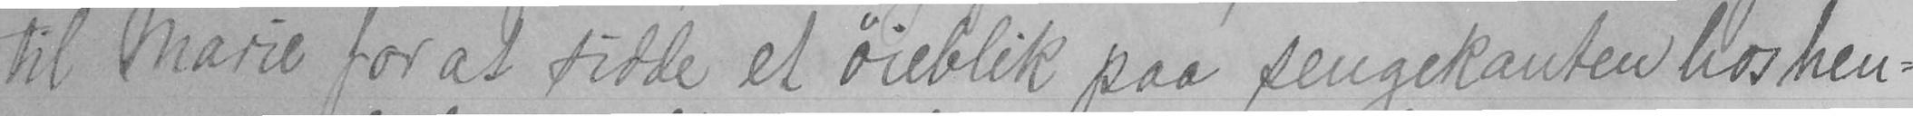til Marie for at sidde et øieblik paa sengekanten hos hen-
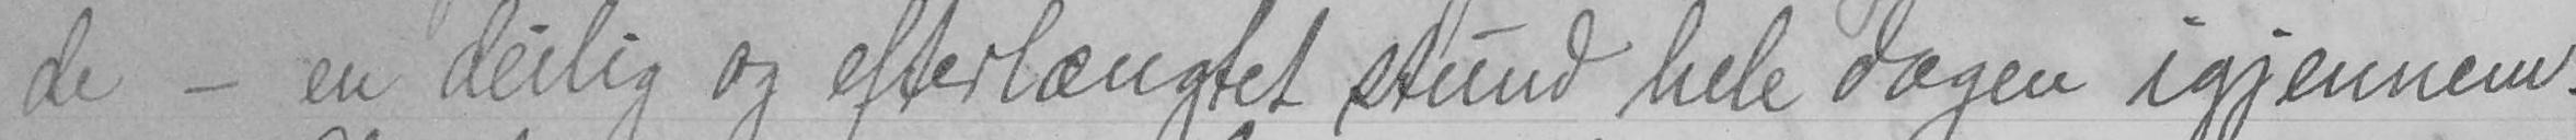de - en deilig og efterlængtet stund hele dagen igjennem.
